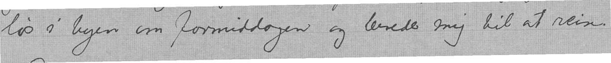løs i byen om formiddagen og bereder mig til at reise.
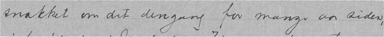snakket om det dengang for mange aar siden,
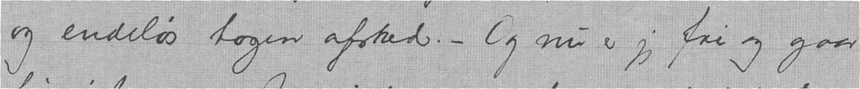og endeløs tagen afsked. — Og nu er jeg fri og gaar
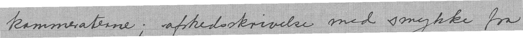kammeraterne; afskedsskrivelse med smykke fra
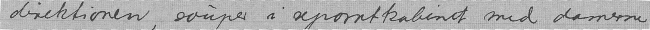direktionen, souper i separatkabinet med damerne
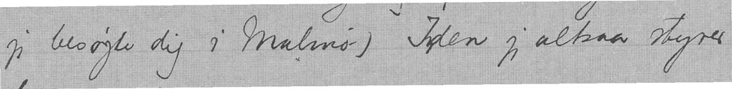jeg besøgte dig i Malmø.) Inden jeg altsaa styrer
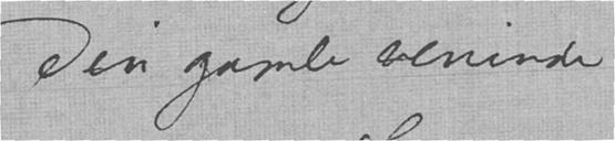Din gamle veninde
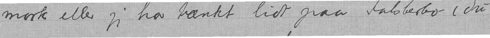mark eller jeg har tænkt lit paa Falsterbo (du
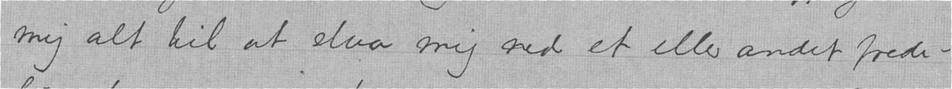mig alt til at slaa mig ned et eller andet frede-
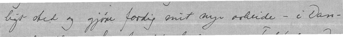ligt sted og gjøre færdig mit nye arbeide — i Dan-
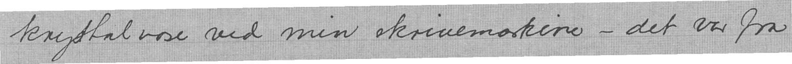krystalvase ved min skrivemaskine — det var fra
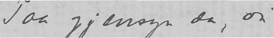Paa gjensyn da, du,
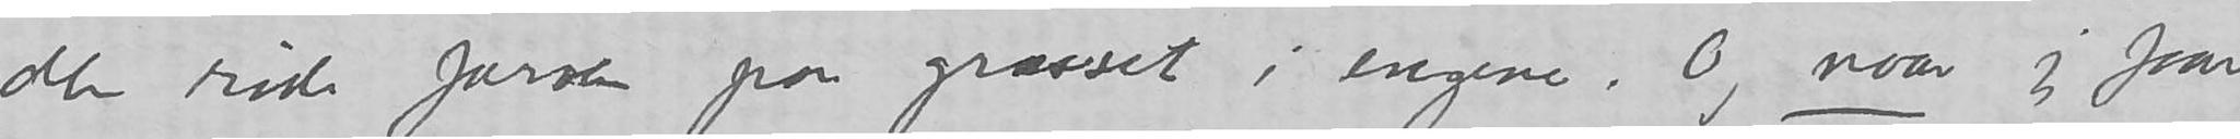røde farven på græsset i engene. Og naar jeg faar
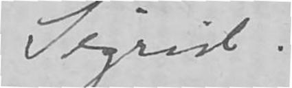Sigrid.
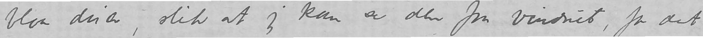blaa druer , slik at jeg kan se dem fra vinduet, for det
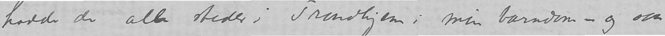hadde de alle steder i Trondhjem i min barndom – og saa
